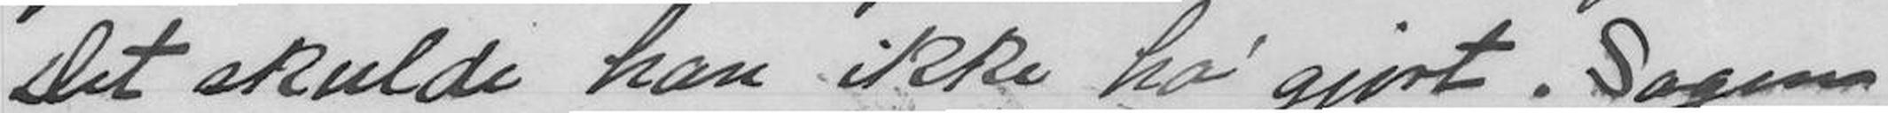Det skulde han ikke ha' gjort. Sagen
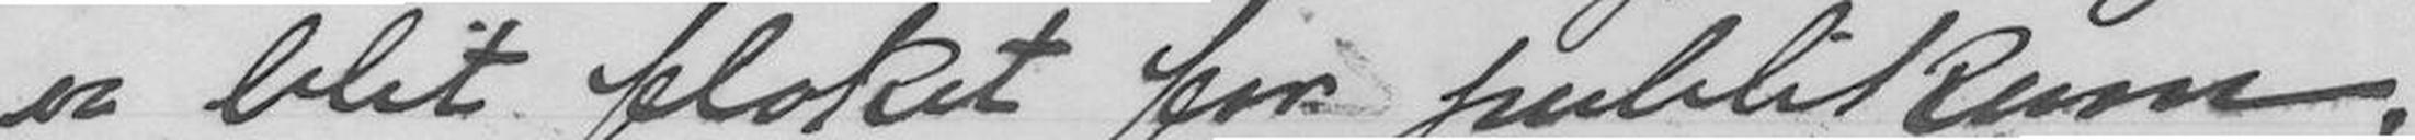er blit floket for publikum,
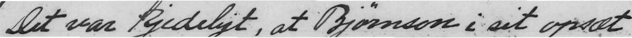Det var kjedeligt, at Bjørnson i sit opsæt
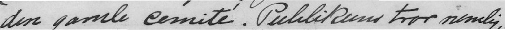den gamle comité. Publikum tror nemlig,
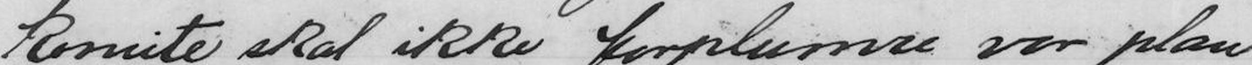komite skal ikke forplumre vor plan.
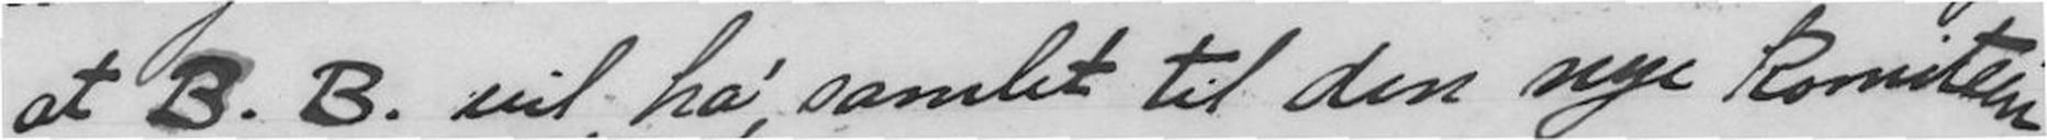at B. B. vil ha' samlet til den nye komitèen,
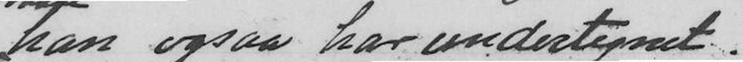af hvilket opraab han ogsaa har undertegnet.
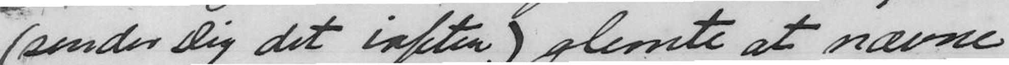(sender Dig det iaften) glemte at nævne
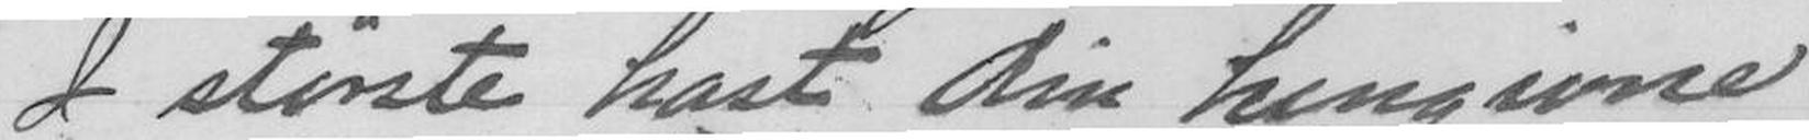I største hast Din hengivne
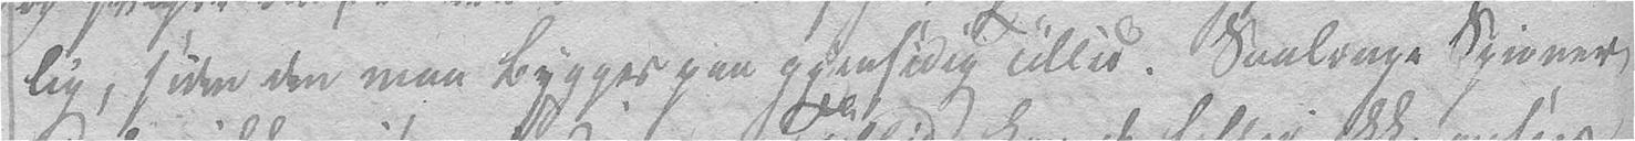lig, siden den maa bygges paa gjensidig Tillid. Saalænge Spioner
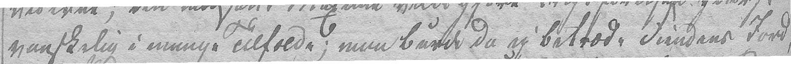vanskelig i mange Tilfælde; man burde da ey betræde Fiendens Jord,
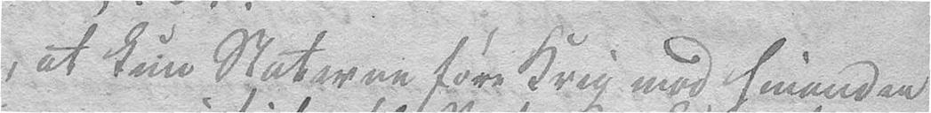, at kun Staterne føre Krig mod hinanden
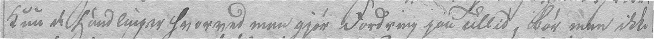Kun de Handlinger hvorved man gjør Fordring paa Tillid, bør man ikke
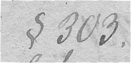§ 303.
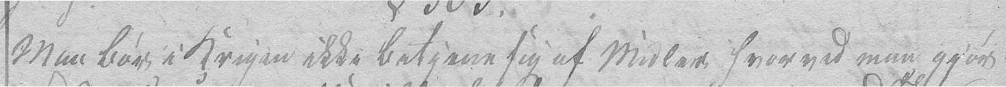Man bør i Krigen ikke betjene sig af Midler hvorved man gjør
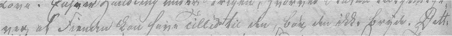ver at Fienden kan have Tillid til den, bør den ikke bryde. Dette
